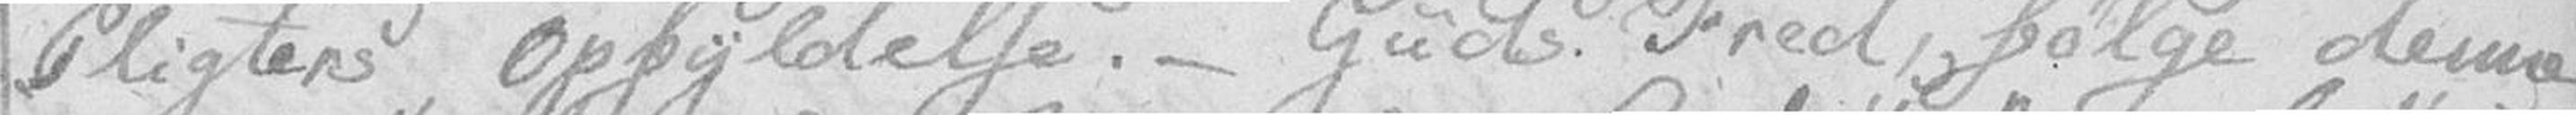Pligters Opfyldelse. – Guds Fred, følge denne
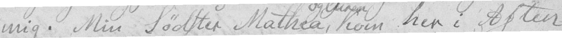mig. Min Sødster Mathea, og Ouren kom her i Aften
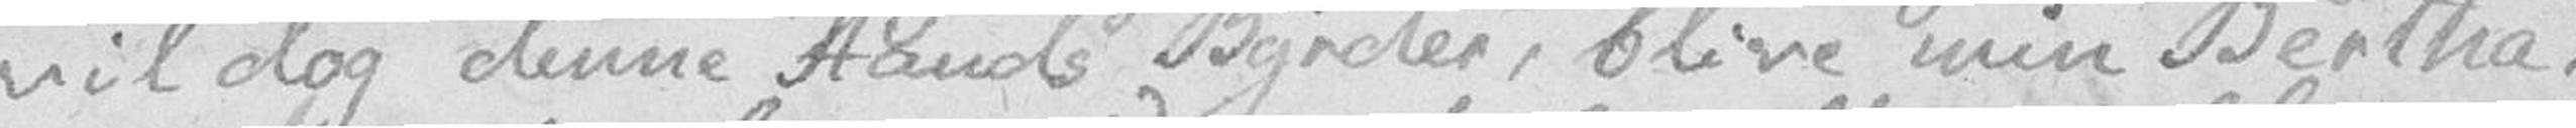vil dog denne Stands Byrder, blive min Bertha!
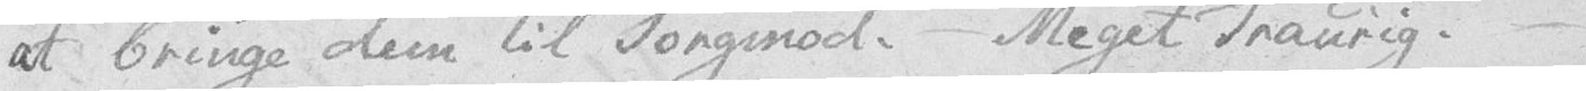at bringe dem til Sorgmod. – Meget Traurig. –
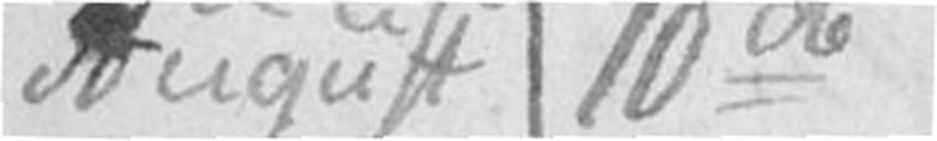August 10de
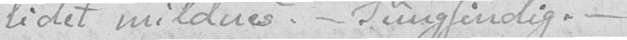lidet mildnes. – Tungsindig. –
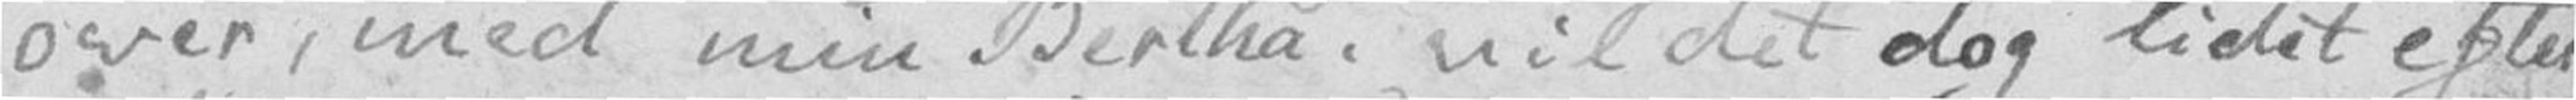over, med min Bertha! vil det dog lidet efter
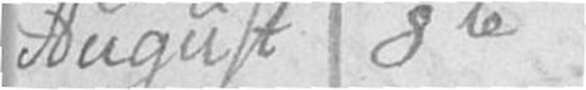August 8te
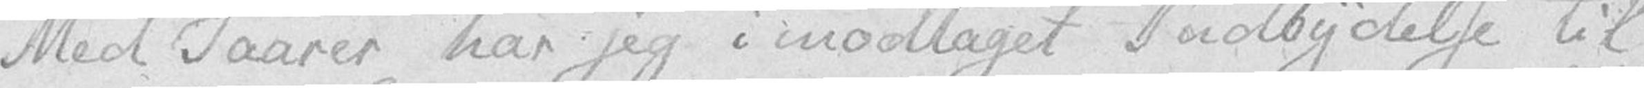Med Taarer har jeg i modtaget Indbydelse til
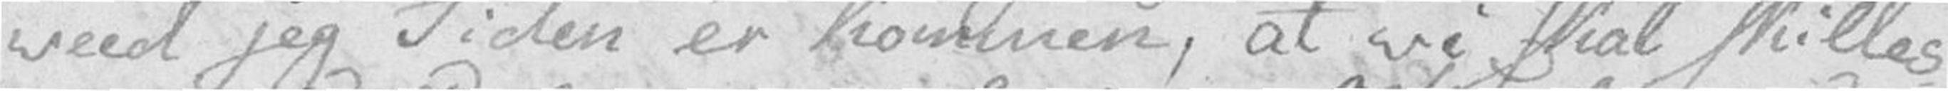veed jeg Tiden er kommen, at vi skal skilles
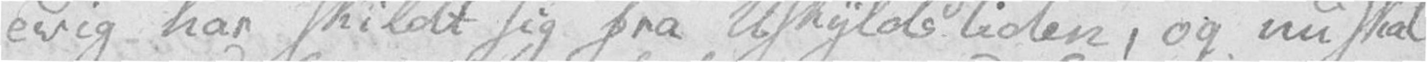evig har skildt sig fra Uskyldstiden, og nu skal
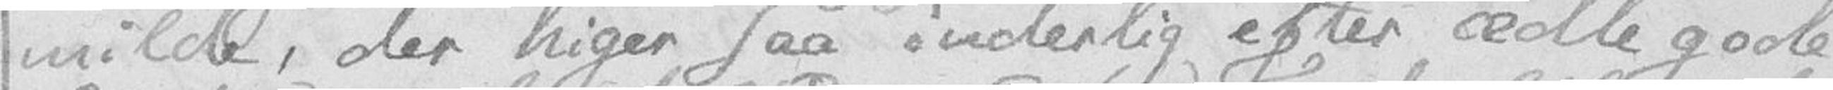milde, der higer saa inderlig efter ædle gode
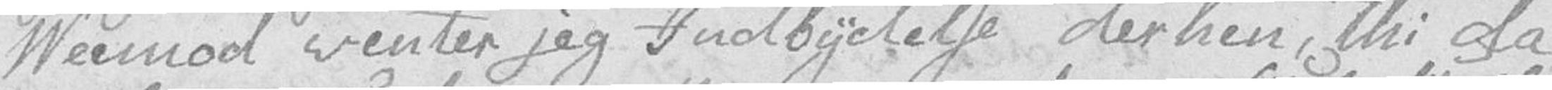Veemod venter jeg Indbydelse derhen, thi da
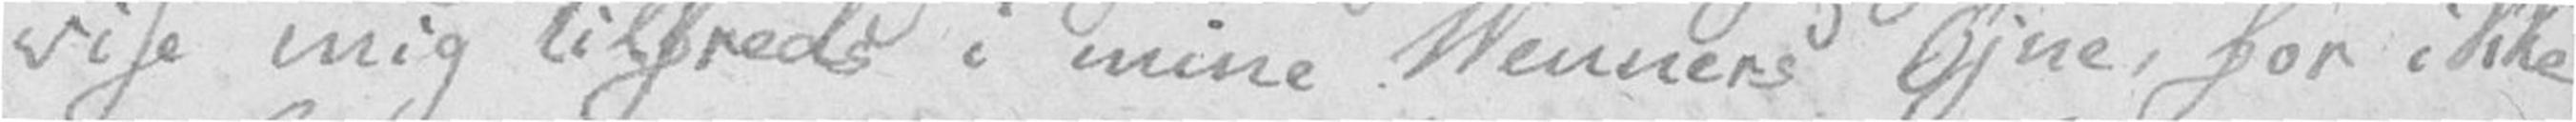vise mig tilfreds i mine Venners Øjne, for ikke
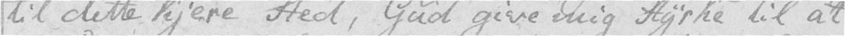til dette kjere Sted, Gud give mig Styrke til at
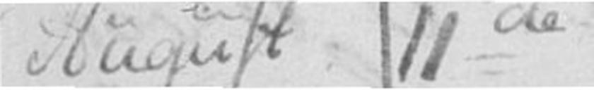August 11de
